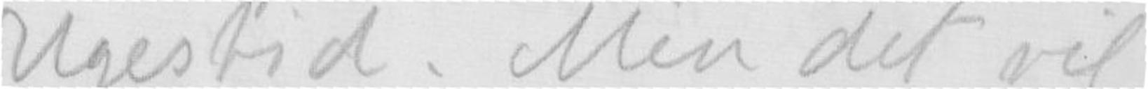Ugestid. Men det vil
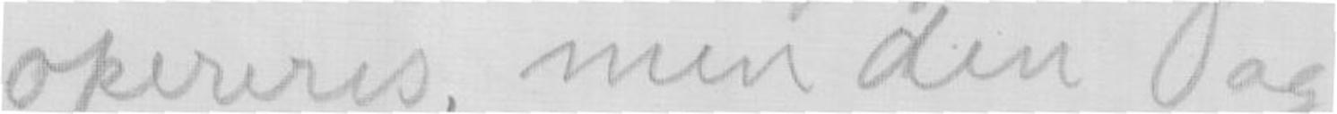opereres, men den Dag
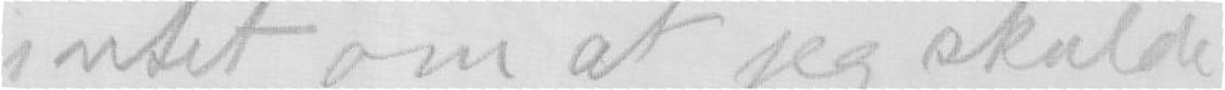intet om at jeg skulde
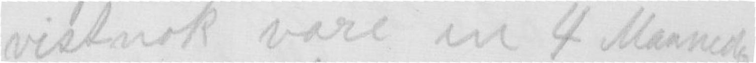vistnok vare en 4 Maaneder
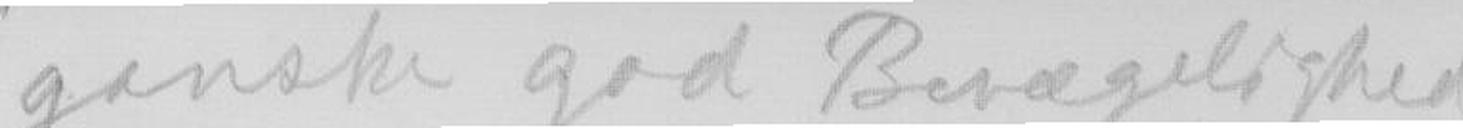ganske god Bevægelighed
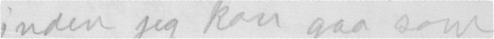inden jeg kan gaa som
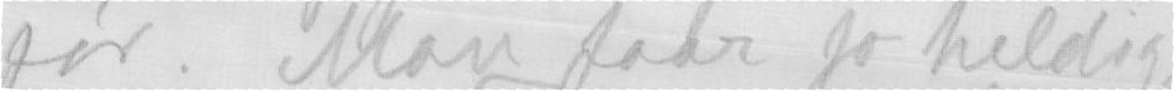før. Man faar jo heldig-
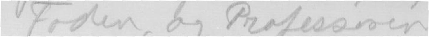Foden, og Professoren
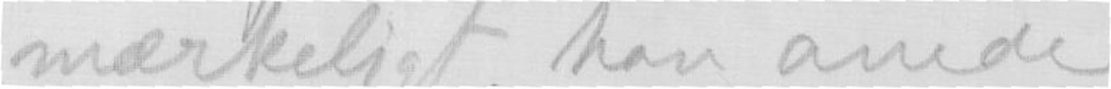mærkeligt, han anede
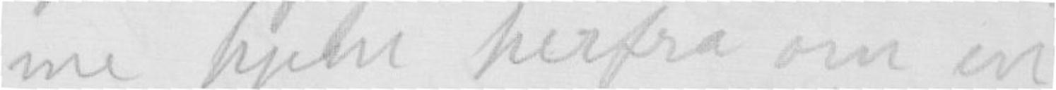me hjem herfra om en
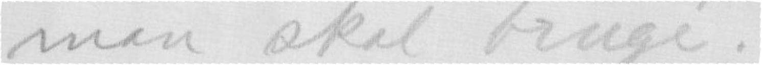man skal bruge.
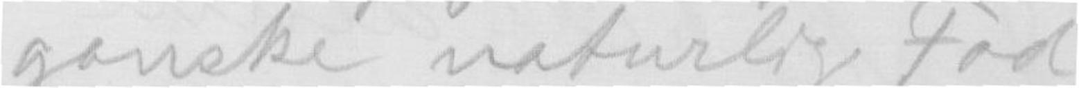ganske naturlig Fod
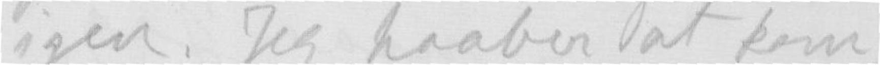igen. Jeg haaber at kom
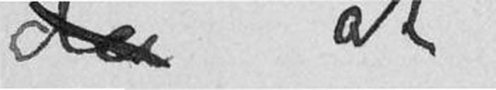da at
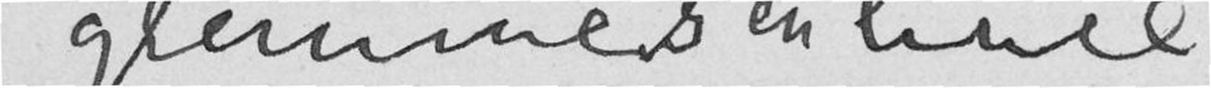glemmes en linie
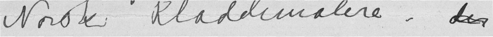Norske Kladdemalere, der
